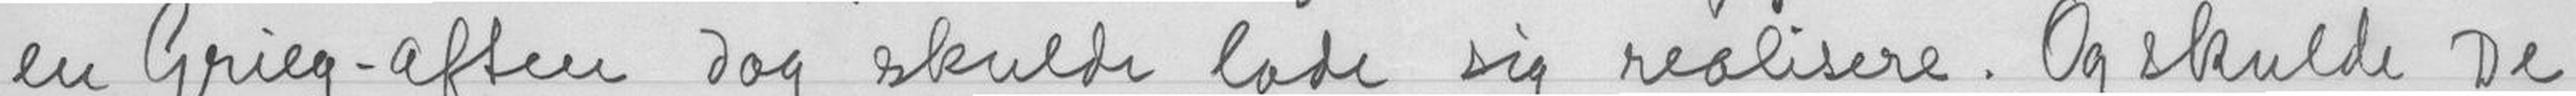en Grieg-aften dog skulde lade sig realisere. Og skulde de
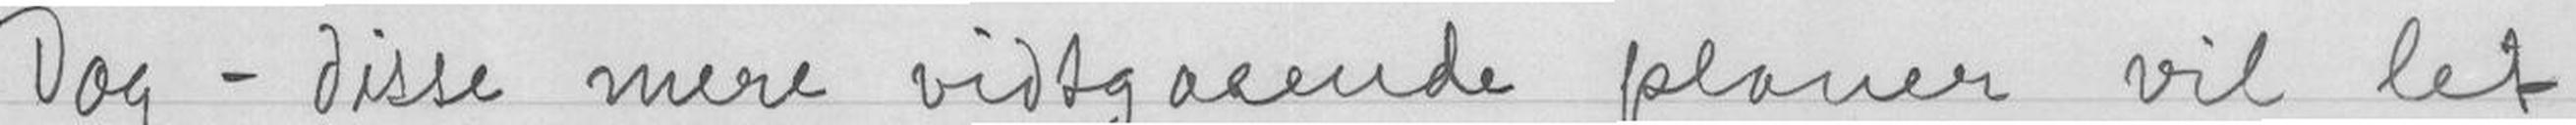Dog - disse mere vidtgaaende planer vil let
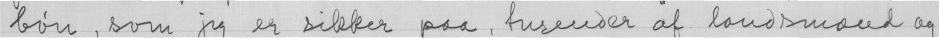bøn, som jeg er sikker paa, tusinder af landsmænd og
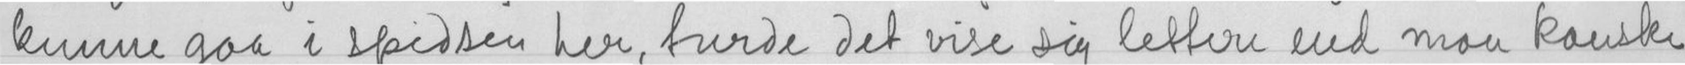kunne gaa i spidsen her, turde det viser sig lettere end man kanske
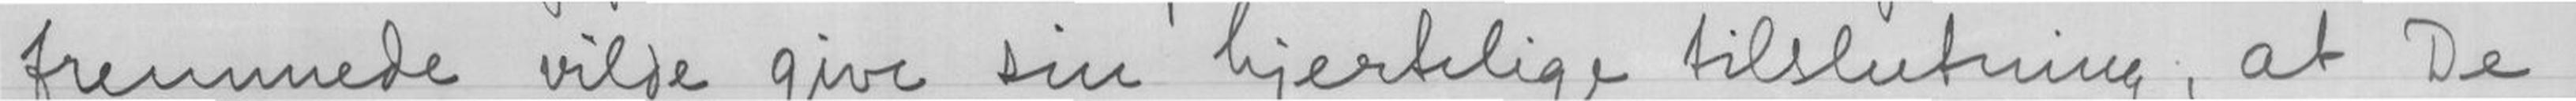fremmede vilde give sin hjertelige tilslutning, at De,
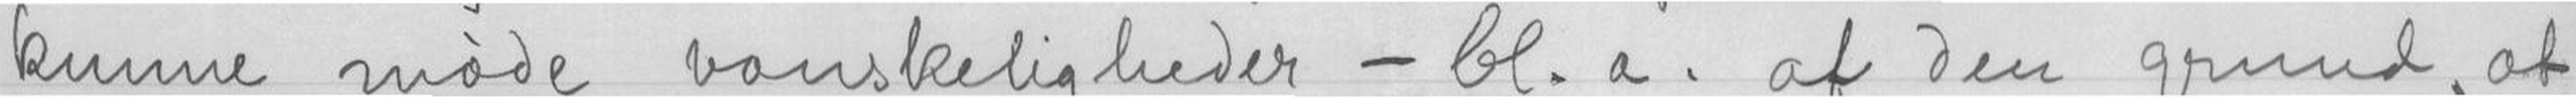kunne møde vanskeligheder - bl. a. af den grund, at
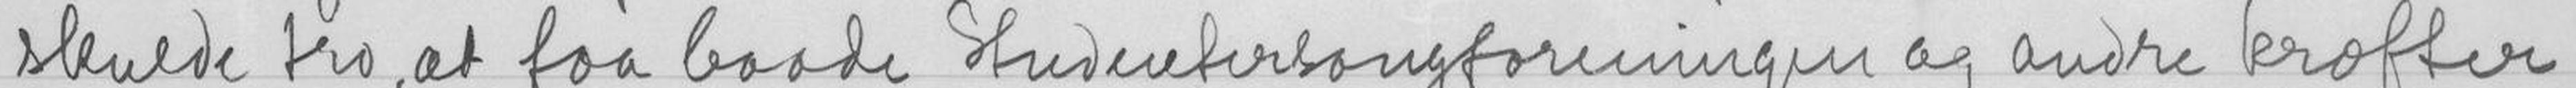skulde tro, at faa baade Studentersongforeningen og andre kræfter
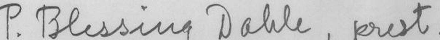P. Blessing Dahle. prest.
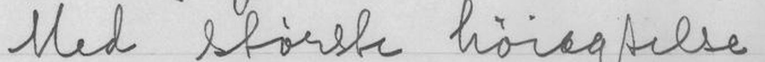Med største høiagtelse
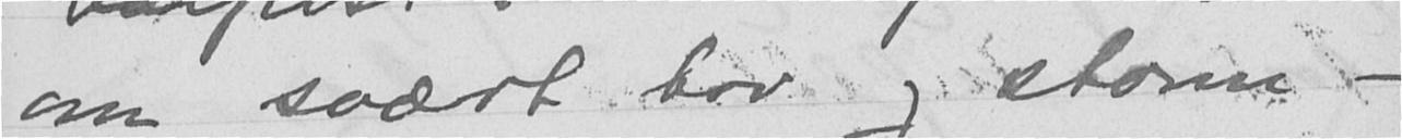om svært kav og storm -
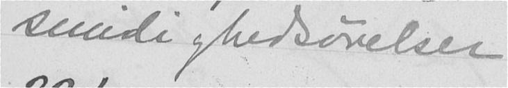smidighedsøvelser
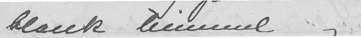blank himmel og
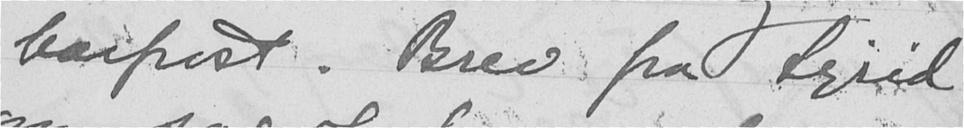barfrost. Brev fra Sigrid
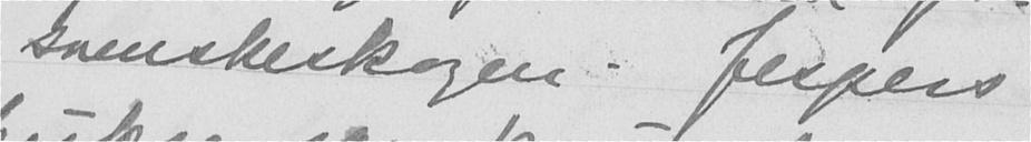svenskeskogen. Jespers
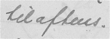til aftens.
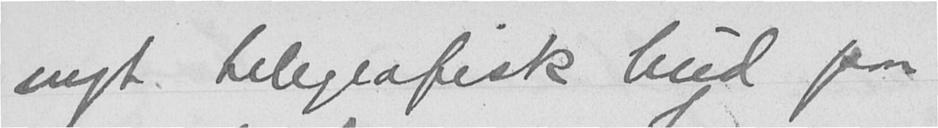nyt telegrafisk bud paa
